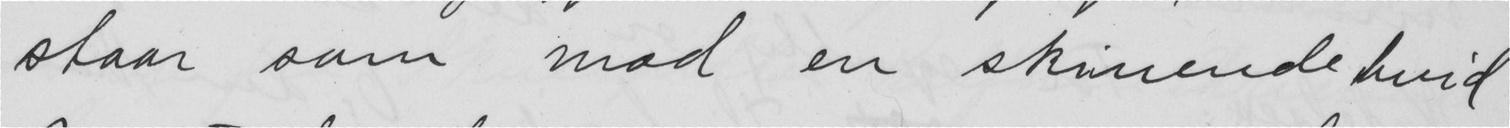staar som mod en skinende hvid
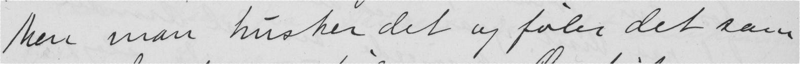Men man husker det og føler det som
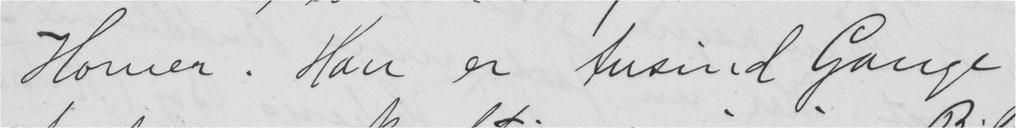Homer. Han er tusind Gange
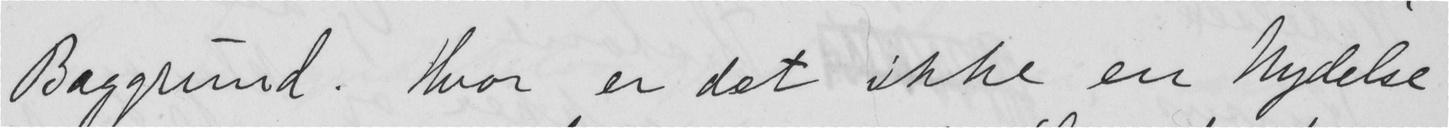Baggrund. Hvor er det ikke en nydelse
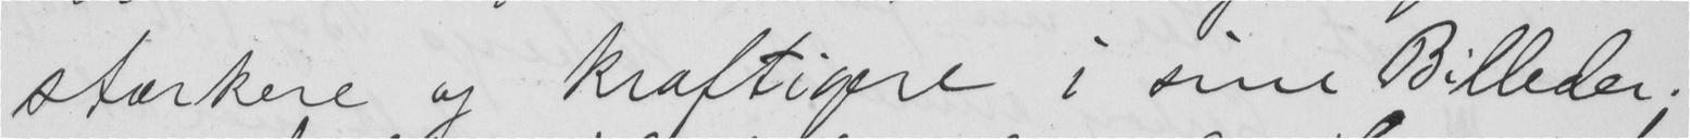stærkere og kraftigere i sine Billeder;
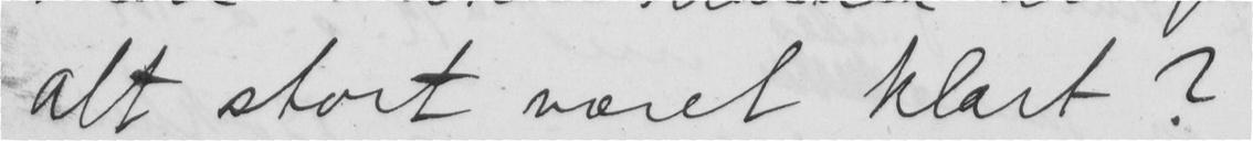alt stort været klart?
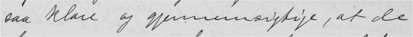saa klare og gjennemsigtige, at de
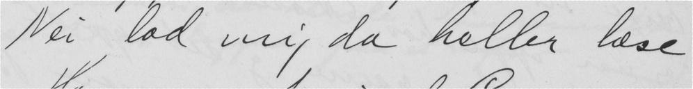Nei lad mig da heller læse
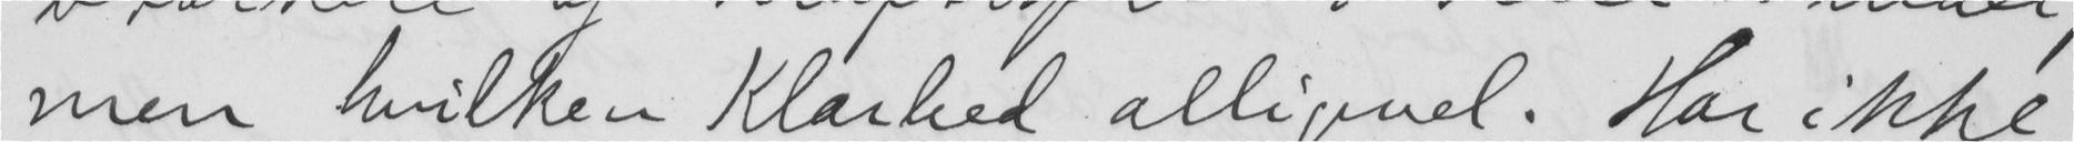men hvilken Klarhed alligevel. Har ikke
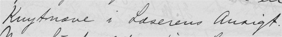Knytnæve i Læserens Ansigt.
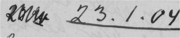23.1.04
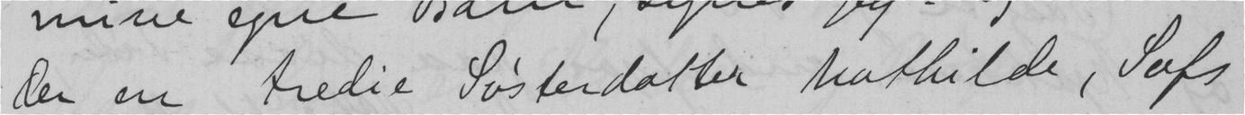der en tredie Søsterdatter Mathilde, Sofs
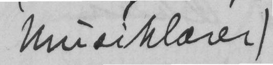musiklærer)
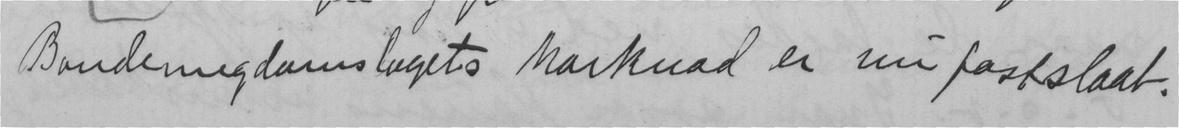Bondeungdomslagets Marknad er nu fastslaat.
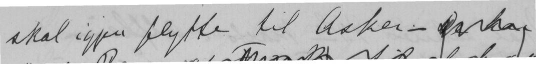skal igjen flytte til Asker -
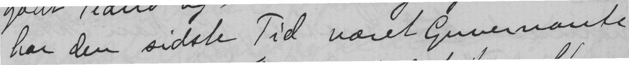har den sidste Tid været Guvernante
